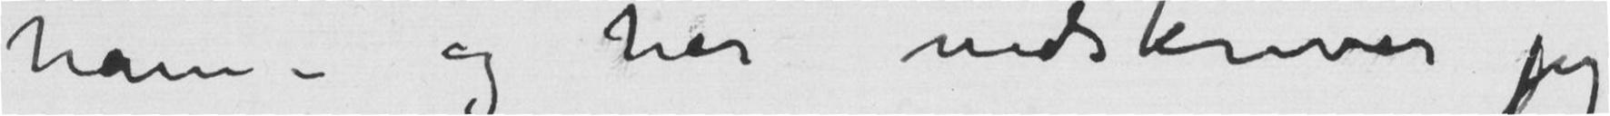ham –	og her nedskriver jeg
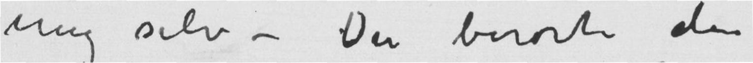mig selv – Du berørte den
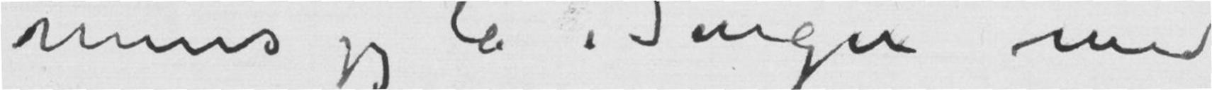mens jeg lå i Sengen med
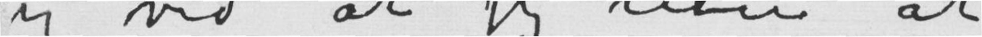jeg ved at jeg uden at
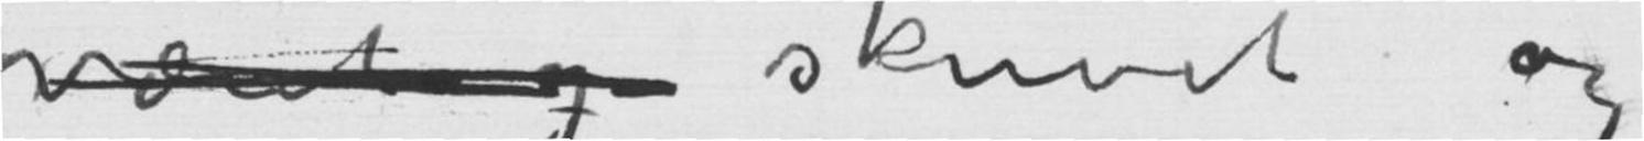og skrevet og
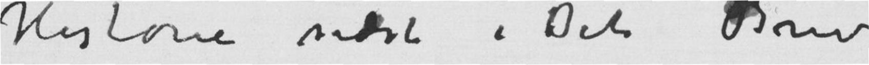Historie sidst i Dit Brev
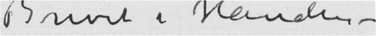Brevet i Hånden –
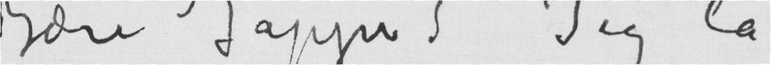Kjære Jappe! Jeg lå
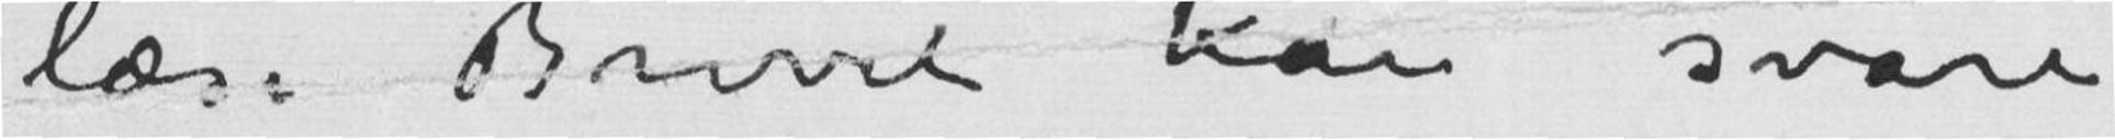læse Brevet kan svare
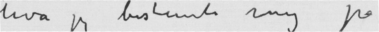hva jeg bestemte mig på
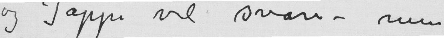og Jappe vil svare – men
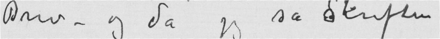Brev – og da jeg så Skriften
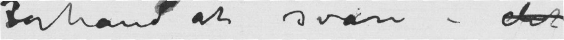Forhaand at svare – det
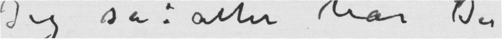Jeg sa: atter har Du
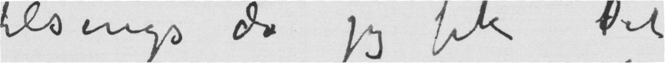tilsengs da jeg fik Dit
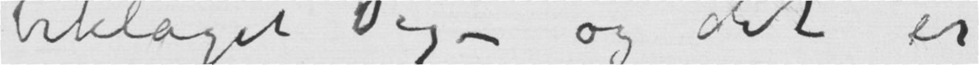beklaget Dig –	og det er
